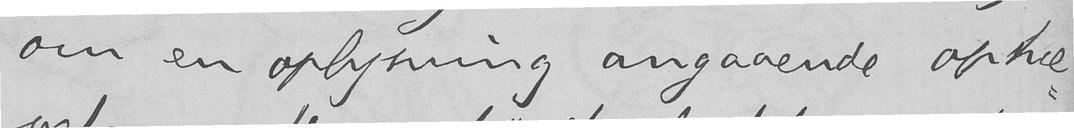om en oplysning angaaende ophæ-
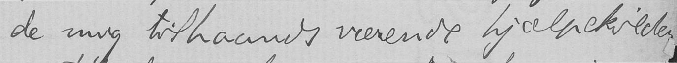de mig tilhaands værende hjælpekilder
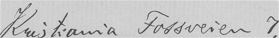Kristiania, Fossveien 7,
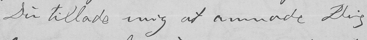Du tillade mig at anmode Dig
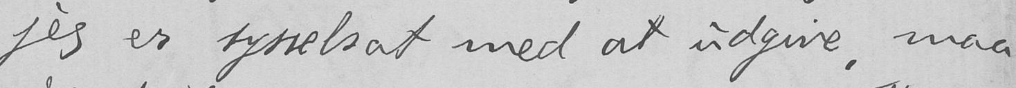jeg er sysselsat med at udgive, maa
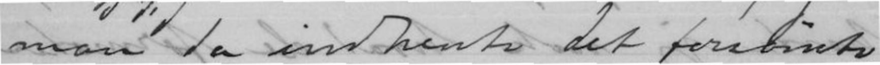man da indhente det forsømte
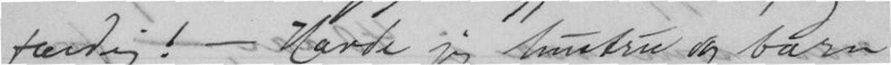færdig! - Havde jeg hustru og barn
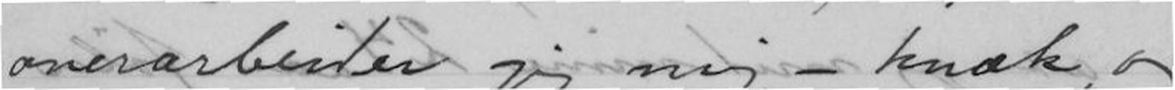overarbeider jeg mig, - knæk, og
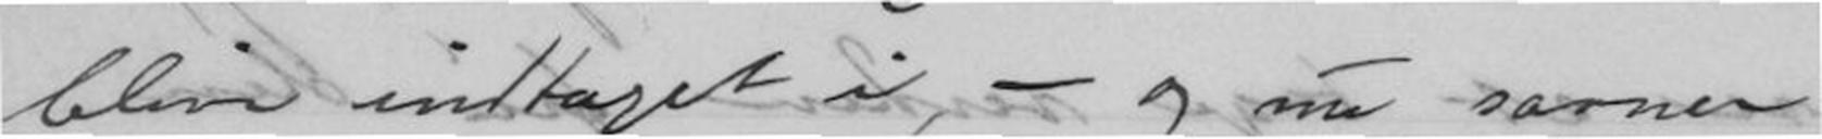blive indtaget i, - og nu savner
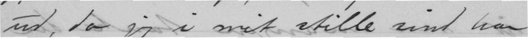ud, da jeg i mit stille sind har
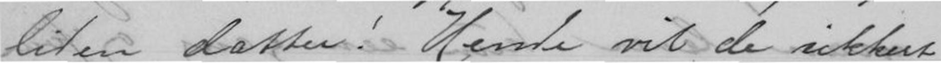liden datter! Hende vil de sikkert
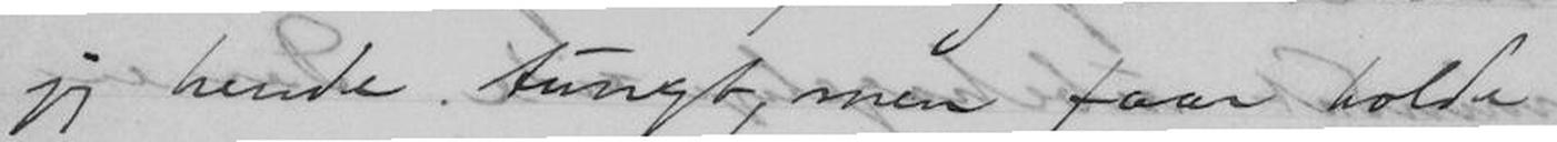jeg hende tungt, men faar holde
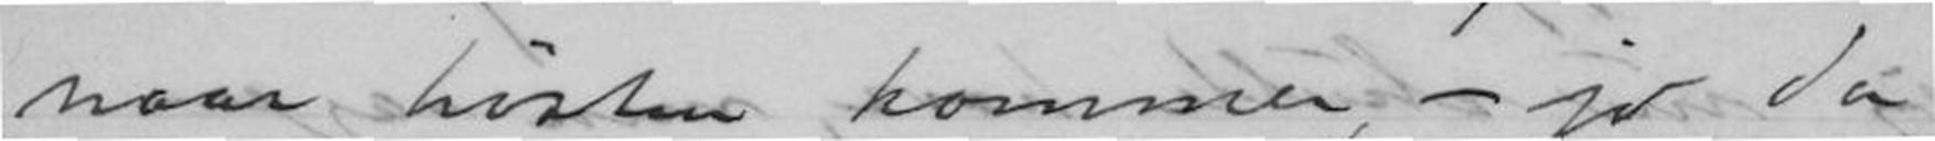naar høsten kommer, - jo da
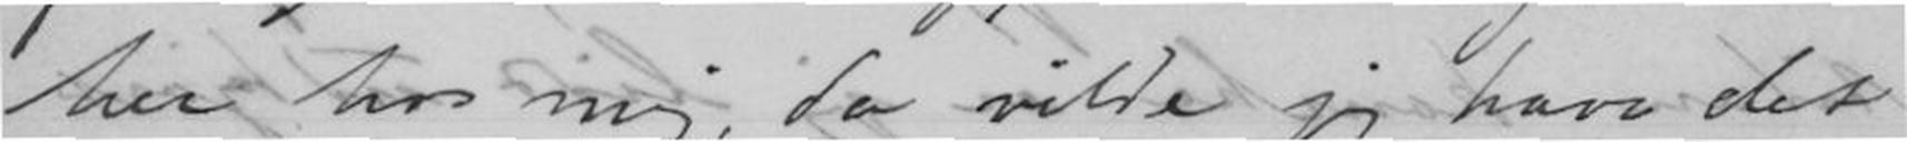her hos mig, da vilde jeg have det
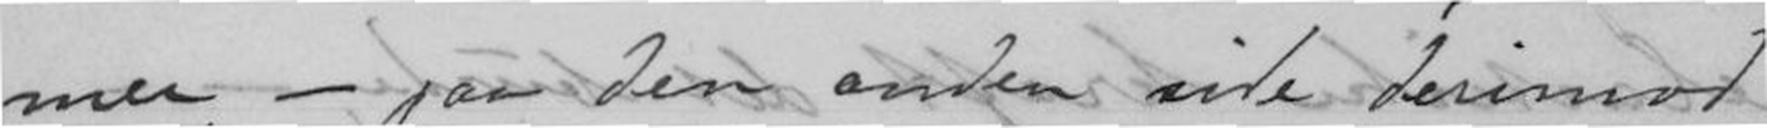mer, - paa den anden side derimod
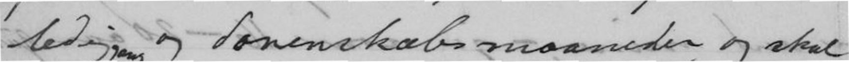lediggang, og dovenskabsmaaneder, og skal
